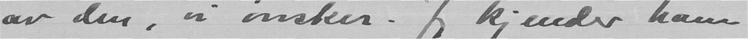av den, vi ønsker. Jeg kjender ham
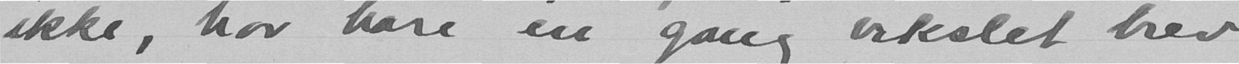ikke, har bare én gang vekslet brev
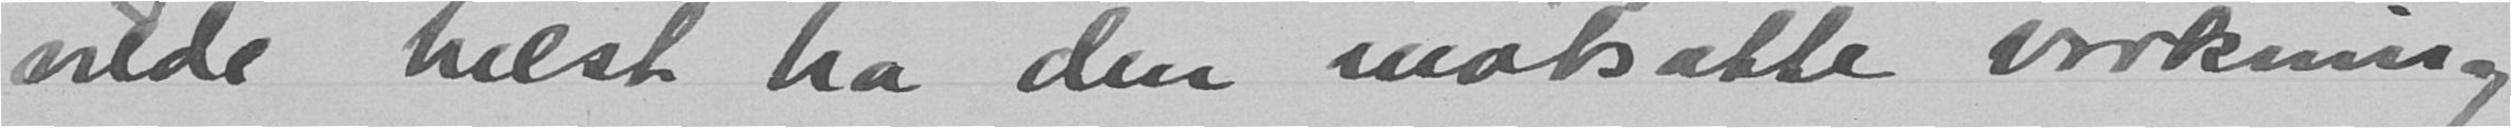vilde helst ga den motsatte virkning
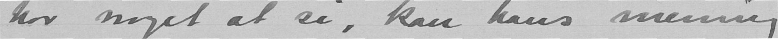har noget at si, kan hans mening
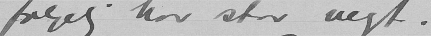følgelig ha stor vegt.
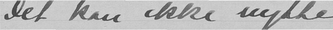Det kan ikke nytte
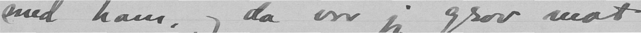med ham, og da var jeg grov mot
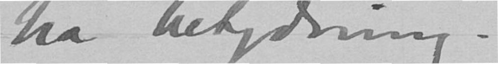ha betydning.
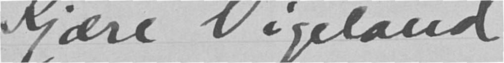Kjære Vigeland
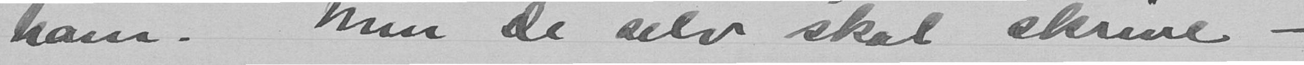ham. Men De selv skal skrive -
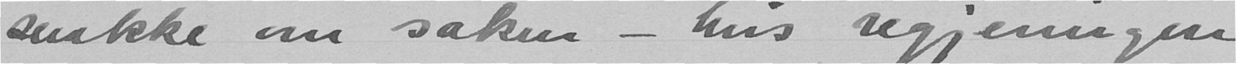snakke om saken - hvis regjeringen
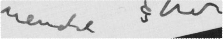uendeli her
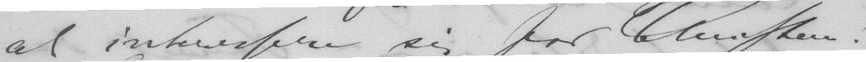at interessere sig for Christen!
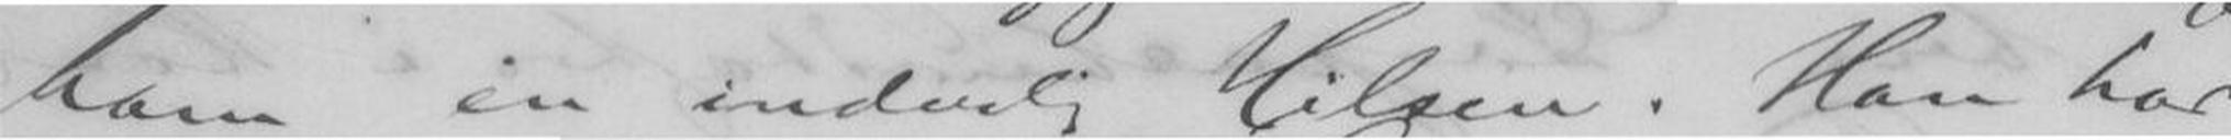ham en inderlig Hilsen. Han har
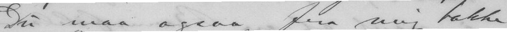Du maa ogsaa fra mig takke
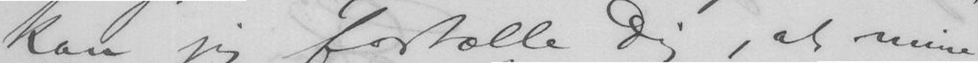kan jeg fortælle Dig, at mine
